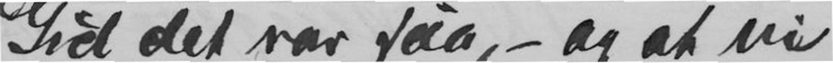Gid det var saa, - og at vi
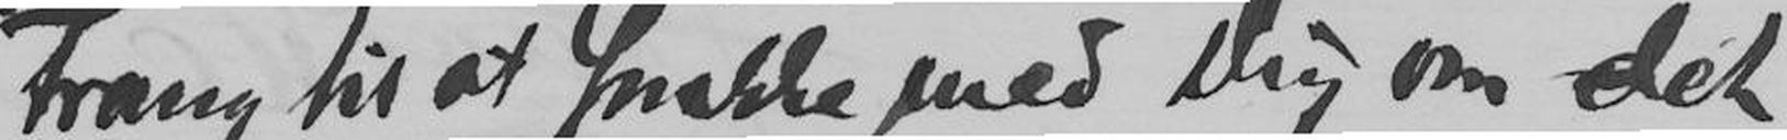Trang til at snakke med Dig om det
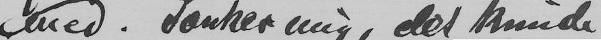med. Tænker mig, det kunde
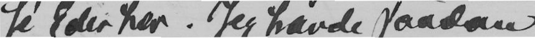se Eder her. Jeg havde saadan
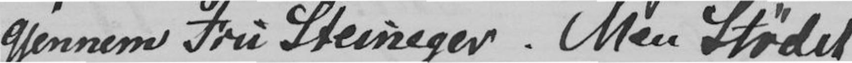gjennem Fru Steineger. Men Stødet
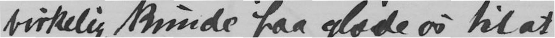virkelig kunde faa glæde os til at
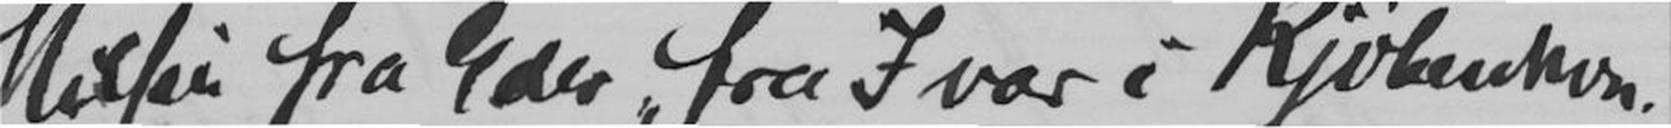Hilsen fra Eder, fra Ivar i Kjøbenhavn.
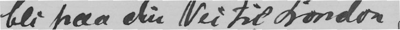bli paa din Vei til London,
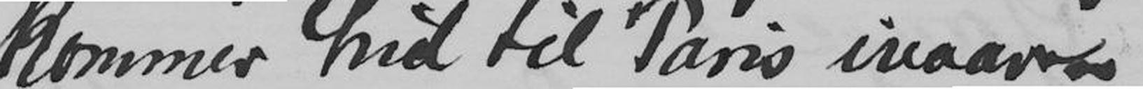kommer hid til Paris ivaares.
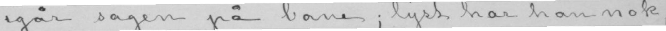igår sagen på bane; lyst har han nok;
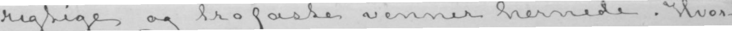rigtige og trofaste venner hernede. Hvor-
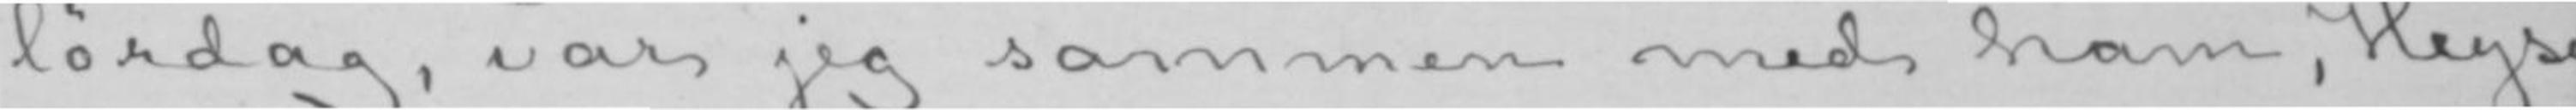lørdag, var jeg sammen med ham, Heyse
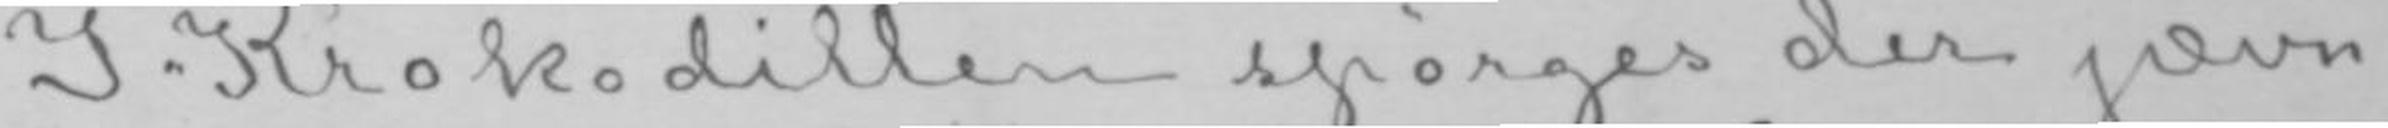I «Krokodillen» spørges der jævn-
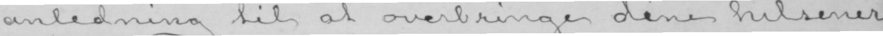anledning til at overbringe dine hilsener
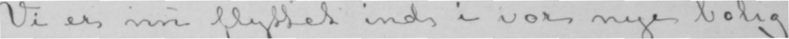Vi er nu flyttet ind i vor nye bolig,
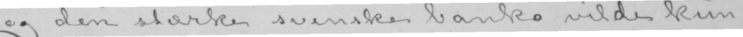og den stærke svenske banko vilde kun-
